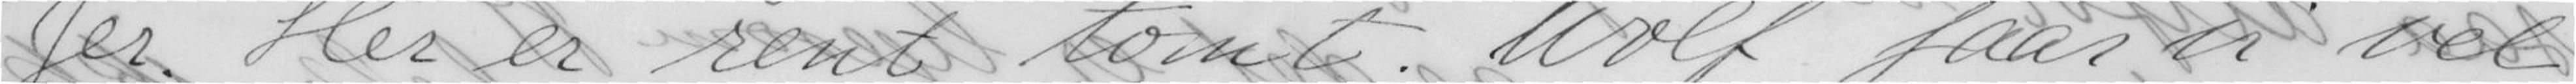Jer. Her er rent tomt. Wolf faar vi vel
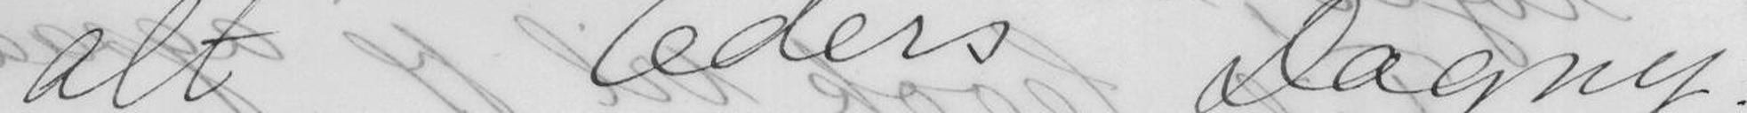alt. Eders Dagny
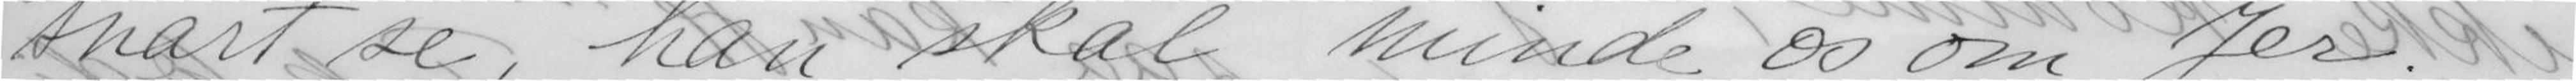snart se, han skal minde os om Jer.
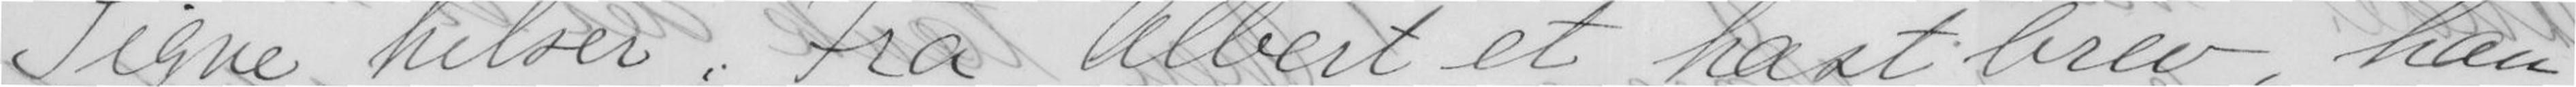Signe hilser. Fra Albert et hast brev, han
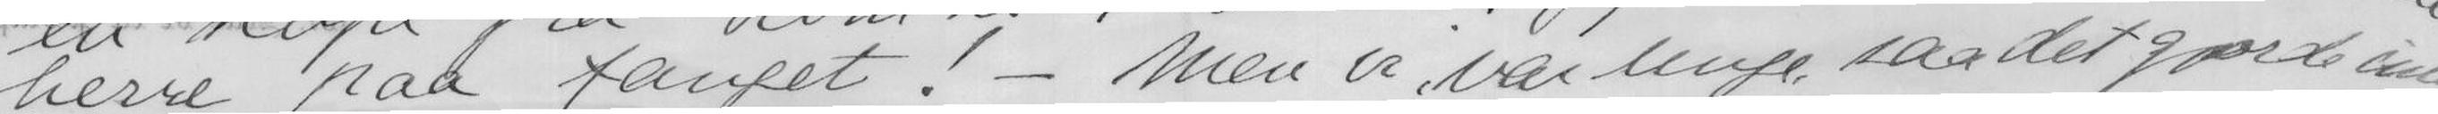herre paa fanget! - Men vi var unge, saa det gjorde intet.
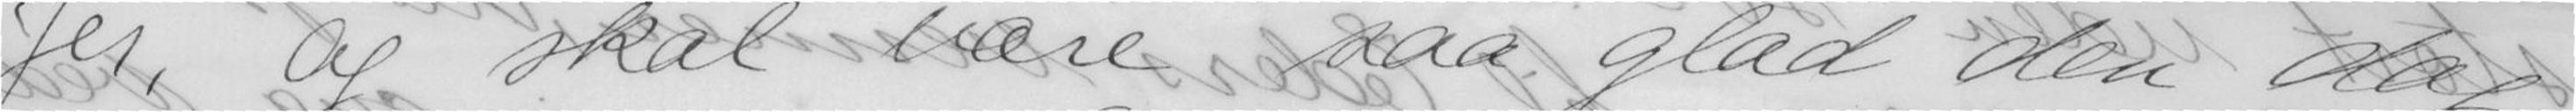Jer, og skal være saa glad den dag
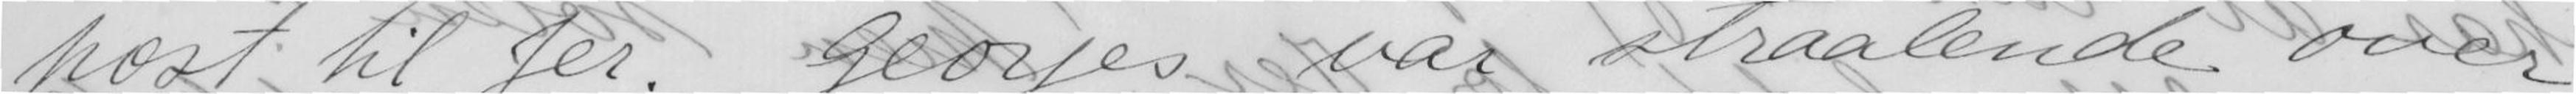post til Jer. Georg var straalende over
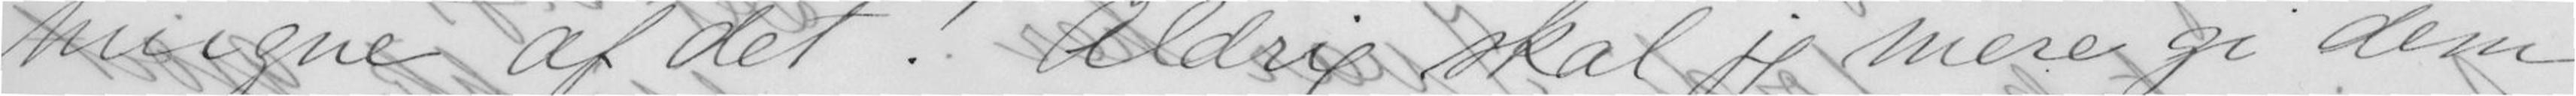mugne af det! Aldrig skal jeg mere gi dem
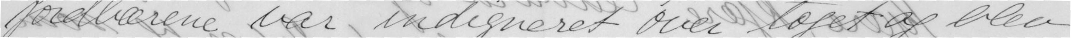jordbærene var indigneret over toget og blev
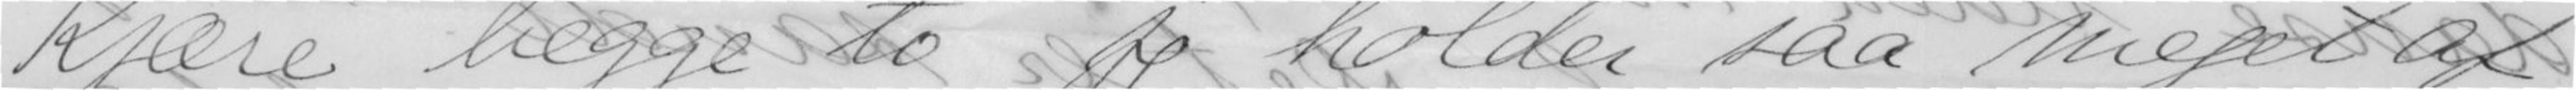Kjære begge to jeg holder saa meget af
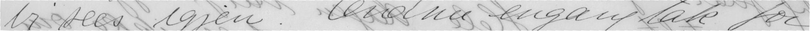vi sees igjen. Endnu engang tak for
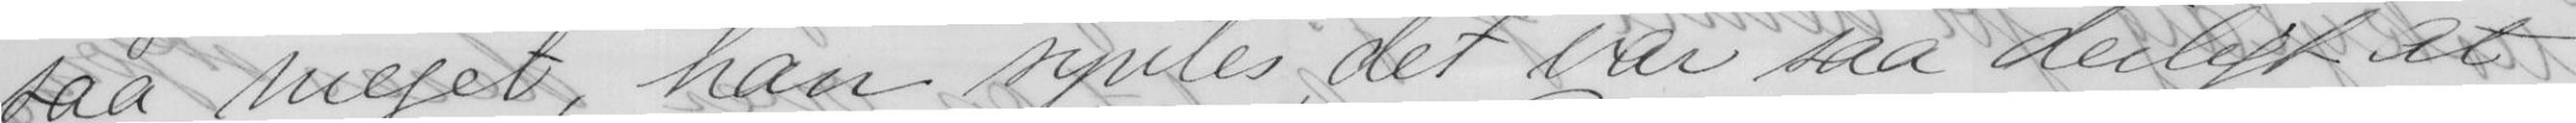saa meget, han syntes det var saa deiligt at
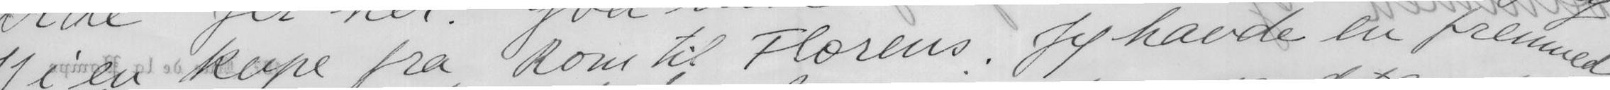11 i en kupe fra Rom til Florens. Jeg havde en fremmed
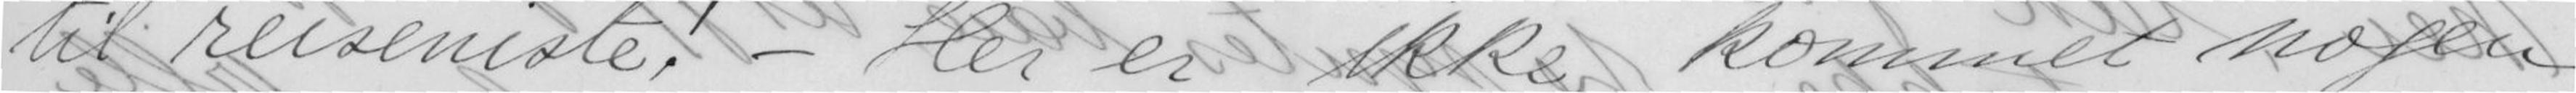til reiseniste! - Her er ikke, kommet nogen
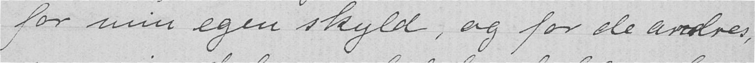for min egen skyld, og for de andres,
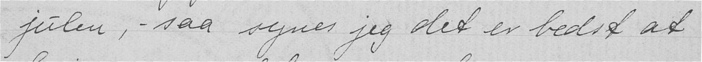julen, - saa synes jeg det er bedst at
